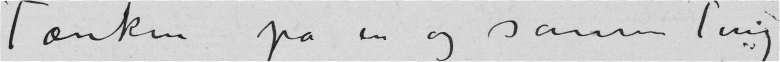Tanken på en og samme Ting –
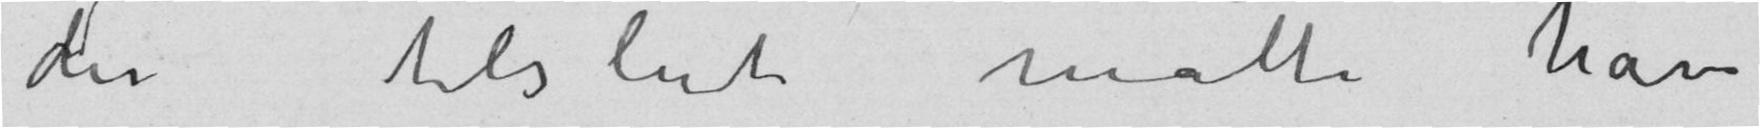der tilslut måtte have
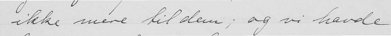ikke mere til dem; og vi havde
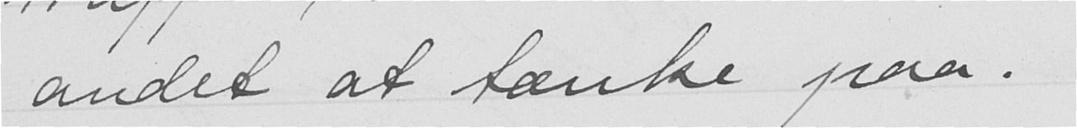andet at tænke paa.
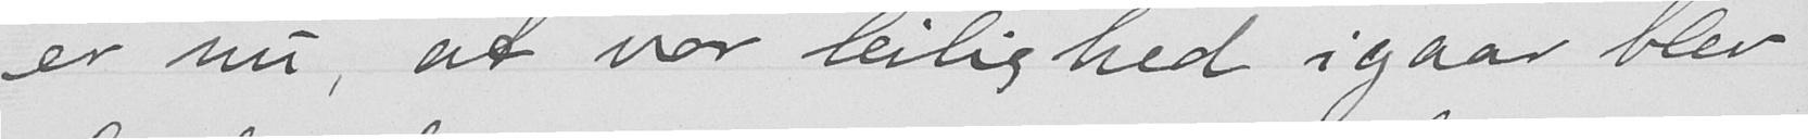er nu, at vor leilighed igaar blev
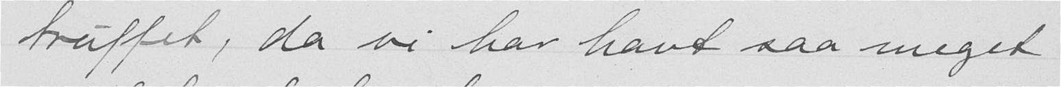truffet, da vi har havt saa meget
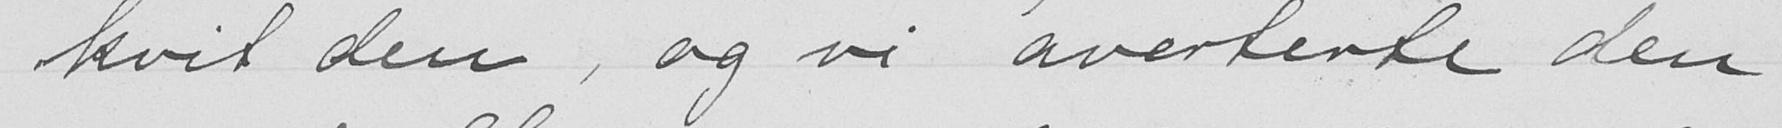kvit den, og vi averterte den
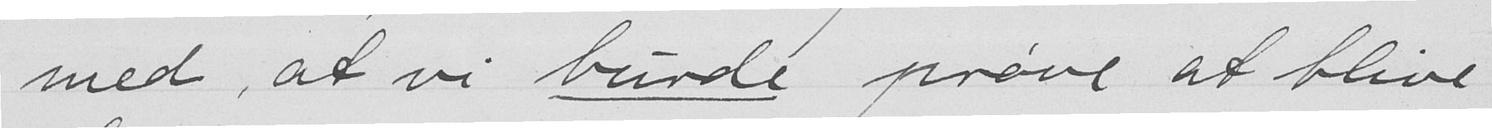med, at vi burde prøve at blive
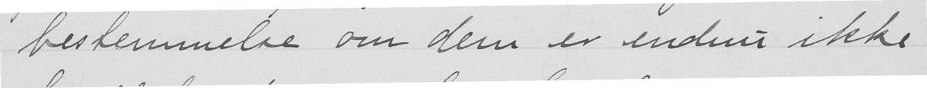bestemmelse om dem er endnu ikke
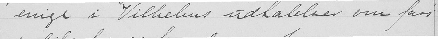enige i Vilhelms udtalelser om fars
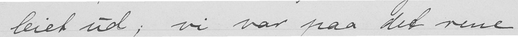leiet ud; vi var paa det rene
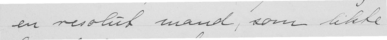en resolut mand, som likte
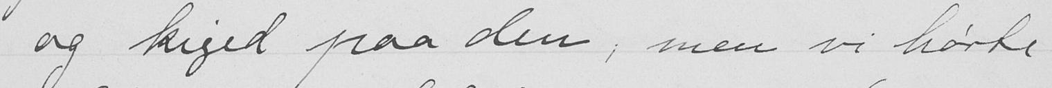og kiged paa den, men vi hørte
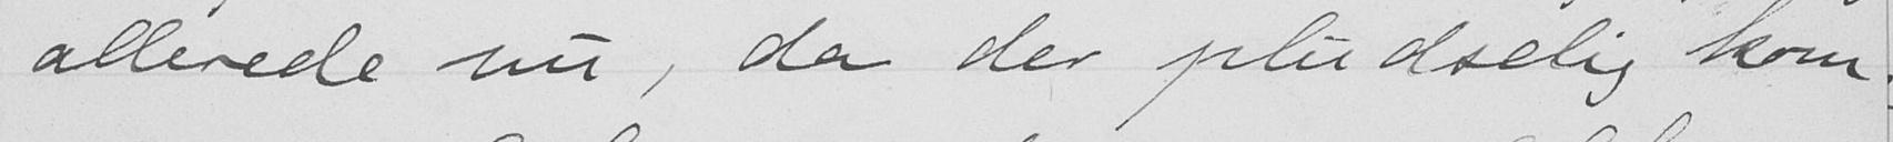allerede nu, da der pludselig kom
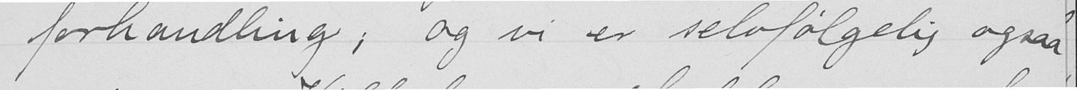forhandling, og vi er selvfølgelig ogsaa
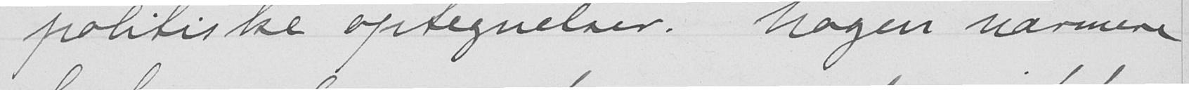politiske optegnelser. Nogen nærmere
